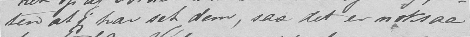ten at jeg har set dem, saa det er noksaa
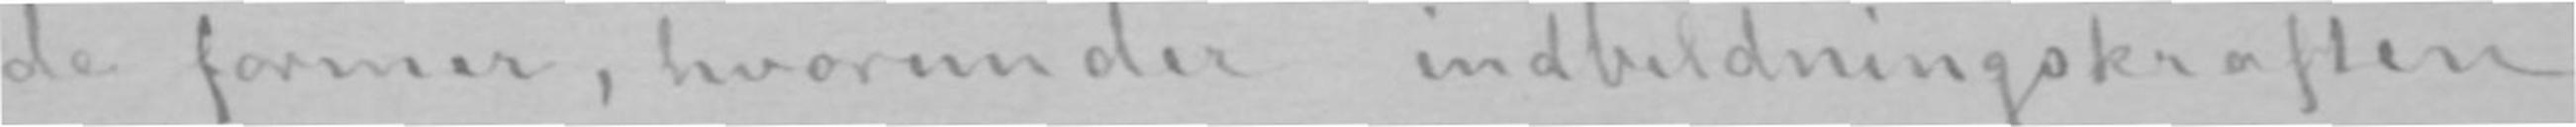de former, hvorunder indbildningskraften
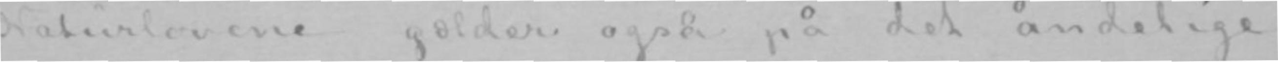Naturlovene gælder også på det åndelige
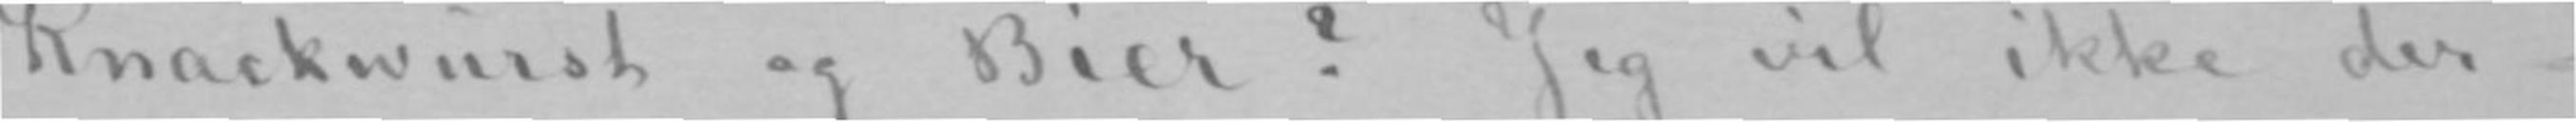Knackwurst og Bier? Jeg vil ikke der-
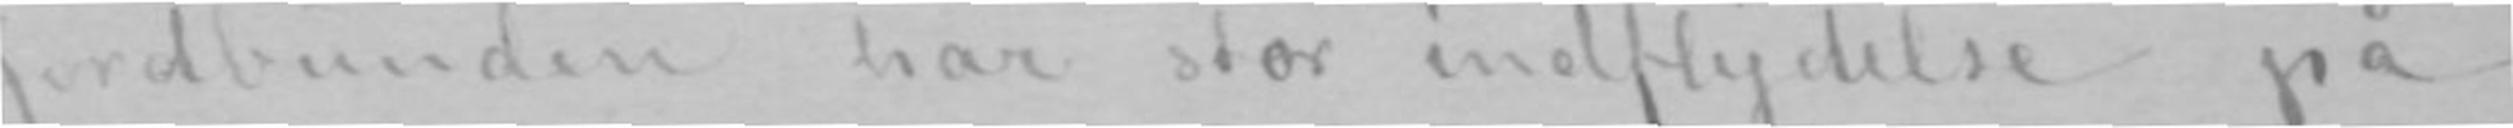Jordbunden har stor indflydelse på
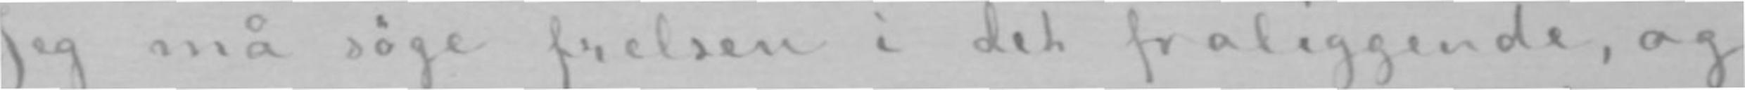Jeg må søge frelsen i det fraliggende, og
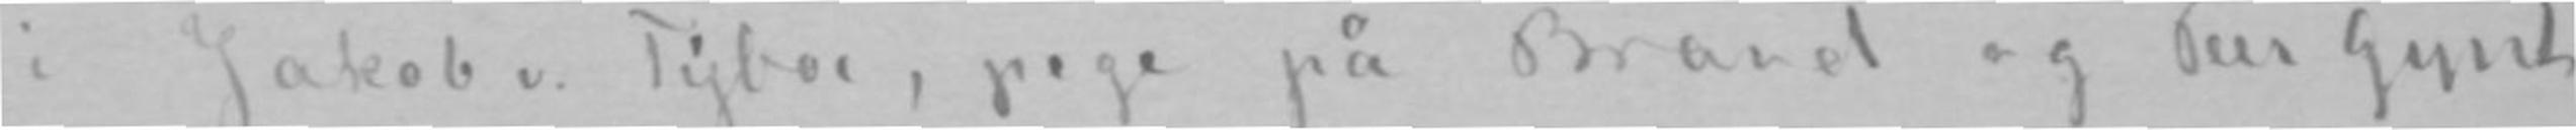i Jakob v. Tyboe, pege på Brand og Peer Gynt
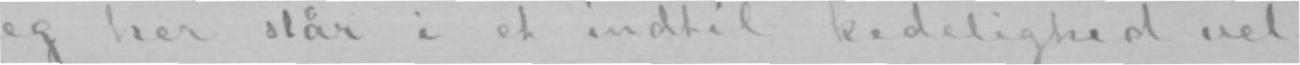jeg her står i et indtil kedelighed vel-
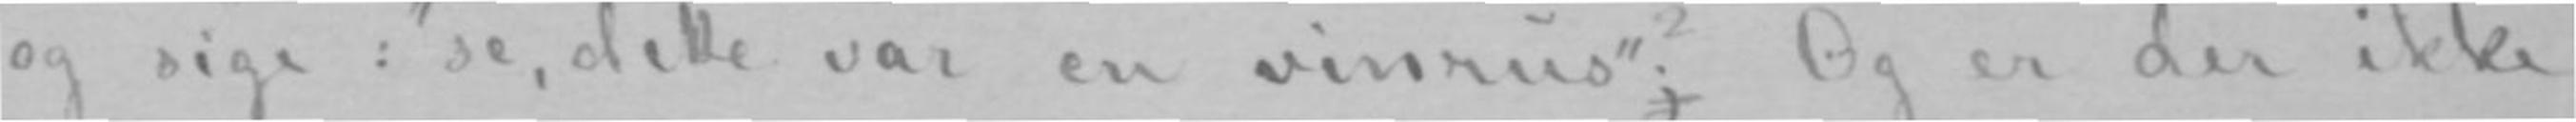og sige: «se, dette var en vinrus»?vinrus»; Og er der ikke
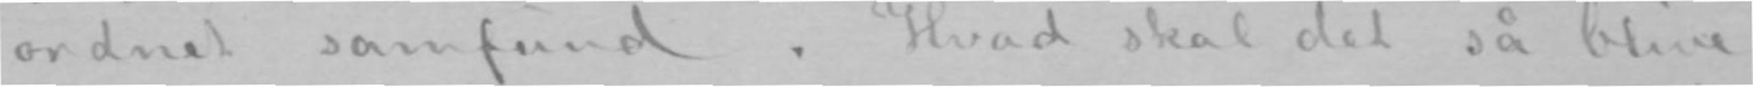ordnet samfund. Hvad skal det så blive
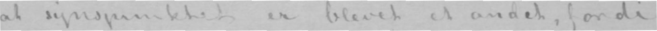at synspunktet er blevet et andet, fordi
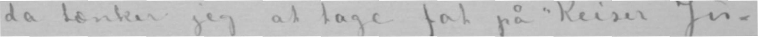da tænker jeg at tage fat på «Keiser Ju-
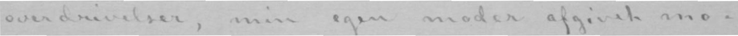overdrivelser, min egen moder afgivet mo-
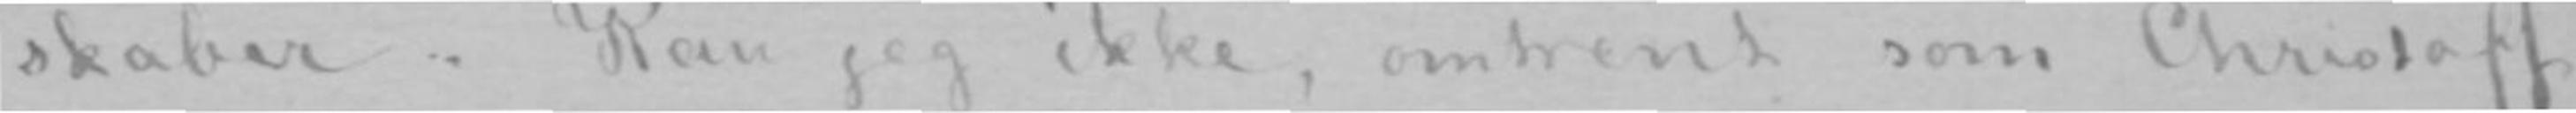skaber. Kan jeg ikke, omtrent som Christoff
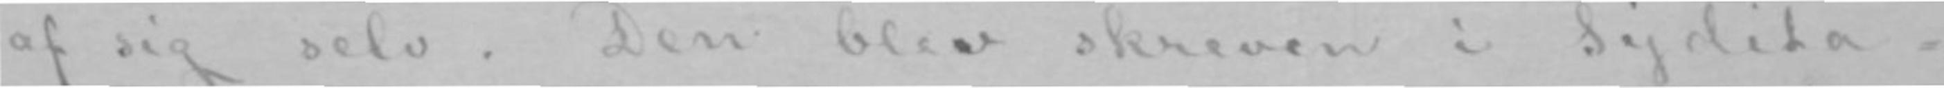af sig selv. Den blev skreven i Sydita-
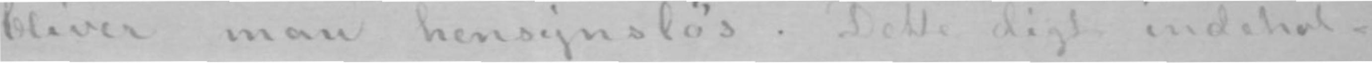bliver man hensynsløs. Dette digt indehol-
